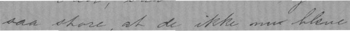saa store, at de ikke mer bleve
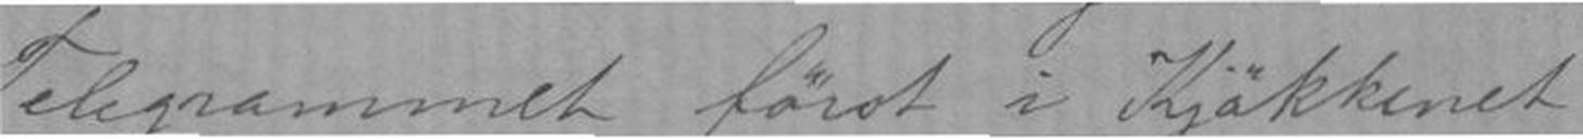Telegrammet først i Kjøkkenet
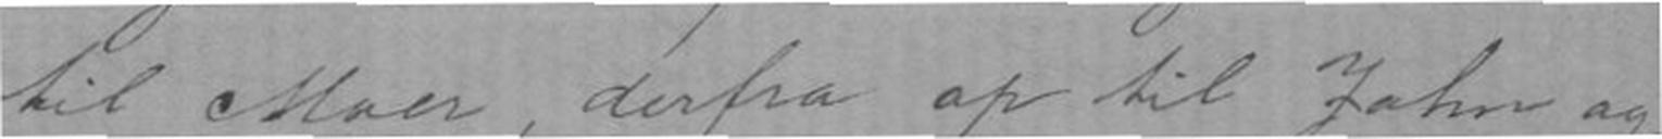til Moer, derfra op til John og
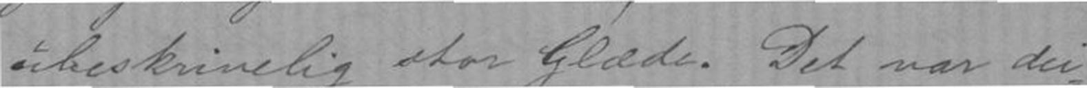ubeskrivelig stor Glæde. Det var dei-
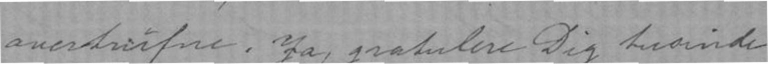overtrufne. Ja, gratulere Dig tusinde
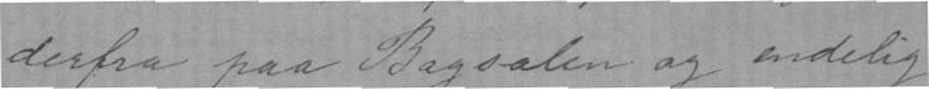Derfra paa Bogsalen og endelig
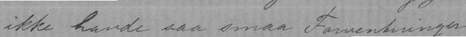ikke have saa smaa Forventninger
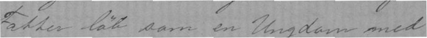Fatter løb som en Ungdom med
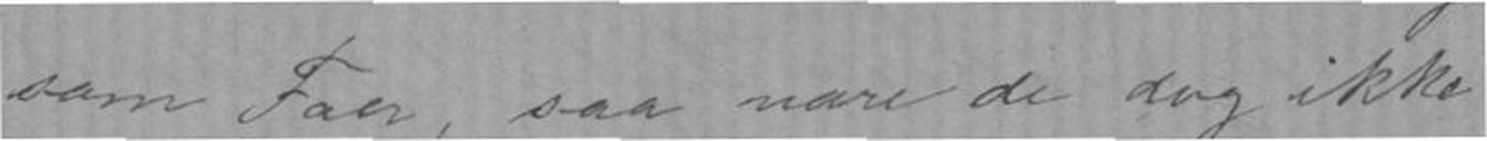som Faer, saa være de dog ikke
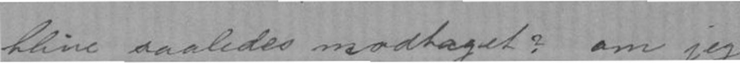blive saaledes modtaget? om jeg
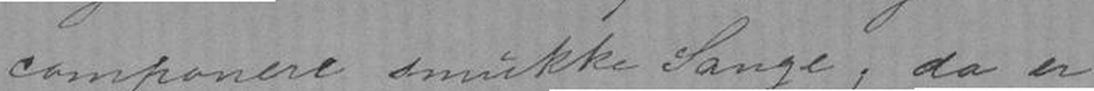componere smukke Sange, da er
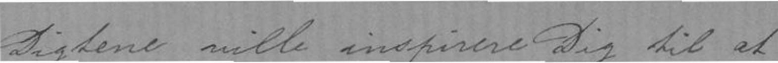Digtene ville inspirere Dig til at
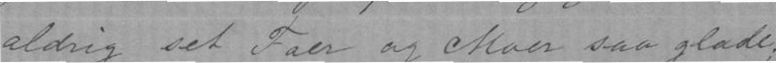aldrig set Faer og Moer saa Glade;
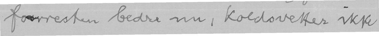forresten bedre nu, koldsvetter ikke
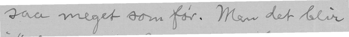saa meget som før. Men det blir
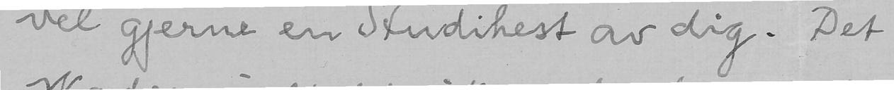vel gjerne en Studihest av dig. Det
